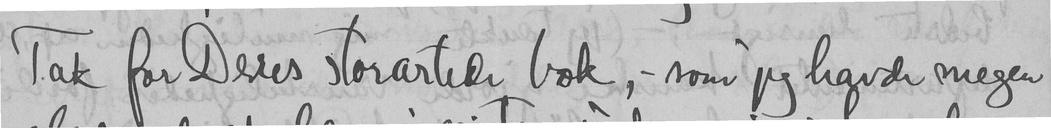Tak for Deres storartede bok, - som jeg havde megen
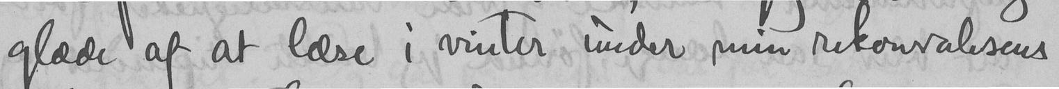glæde af at læse i vinter under min rekonvalesens
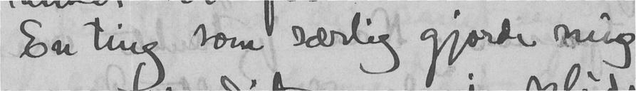En ting som særlig gjorde mig
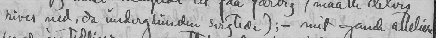rives ned, da undergrunden svigtede); - mit gamle atteliere
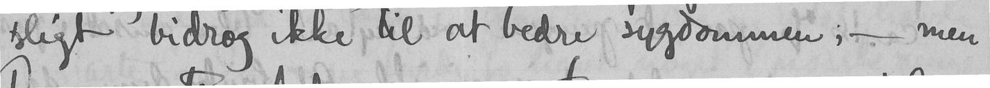sligt bidrog ikke til at bedre sygdommen; - men
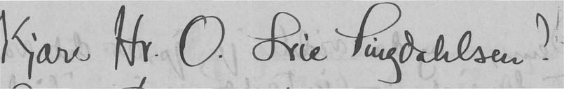Kjære Hr. O. Lie Singdahlsen!
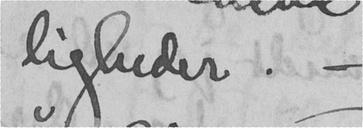ligheder. -
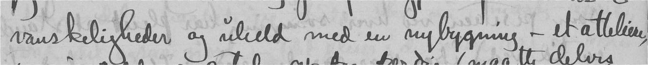vanskeligheder og uheld med en nybygning - et atteliere,
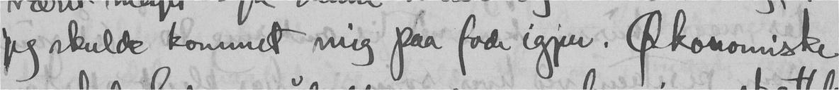jeg skulde kommet mig paa fode igjen. Økonomiske
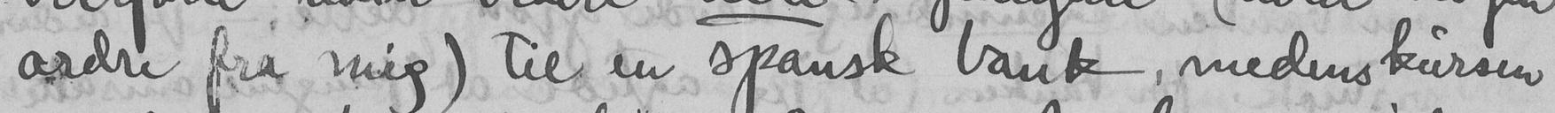ordre fra mig) til en spansk bank, medens kursen
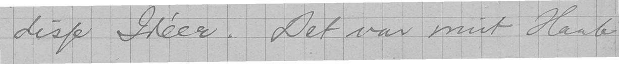disse Ideer. Det var mit Haab,
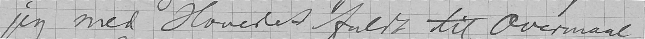jeg med Hovedet fuldt til Overmaal
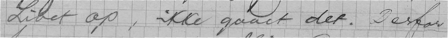Livet op, ikke gaaet det. Derfor
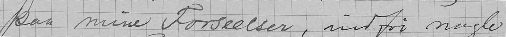paa mine Forseelser, indfri nogle
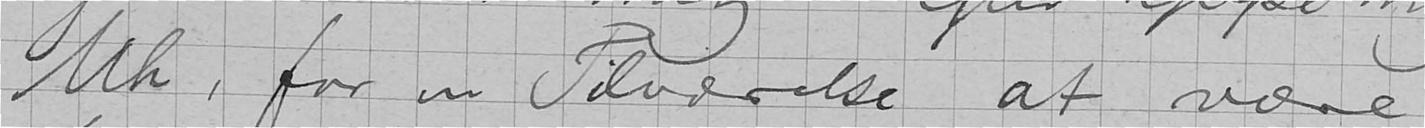Uh, for en Tilværelse at være
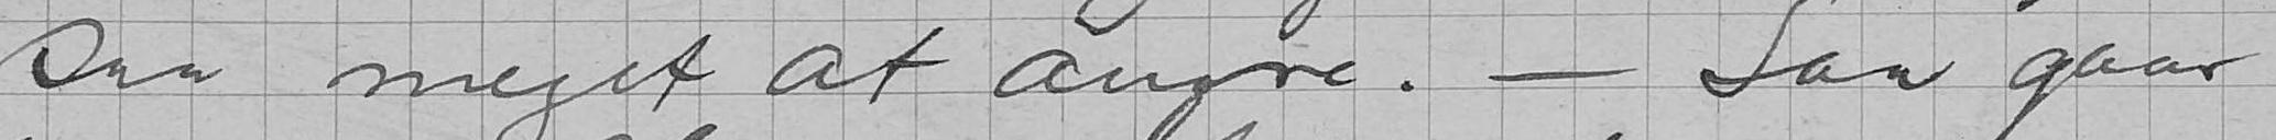saa meget at angre. - Saa gaar
